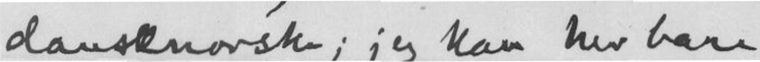dansknorske; jeg kan her bare
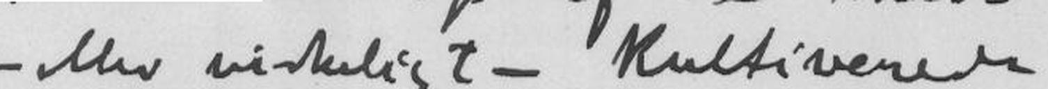- eller virkeligt - kultiverede
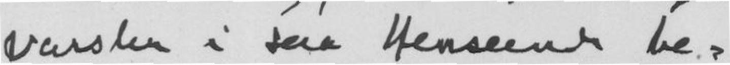Varsler i saa Henseende be-
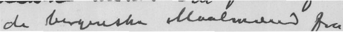de bergenske Maalmænd fra
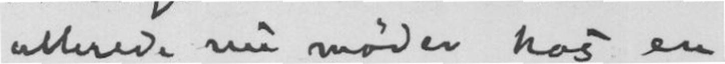allerede nu møder hos en
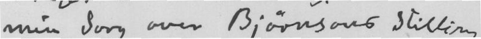min Sorg over Bjørnsons Stilling
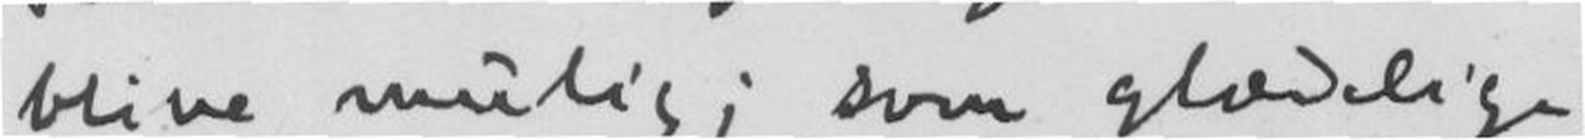blive mulig; som glædelige
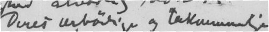Deres ærbødige og taknemmelige
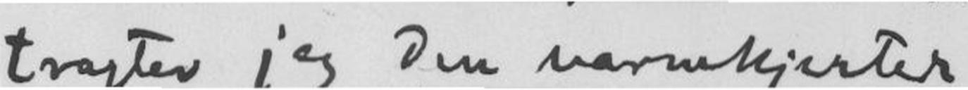tragter jeg den varmhjertede
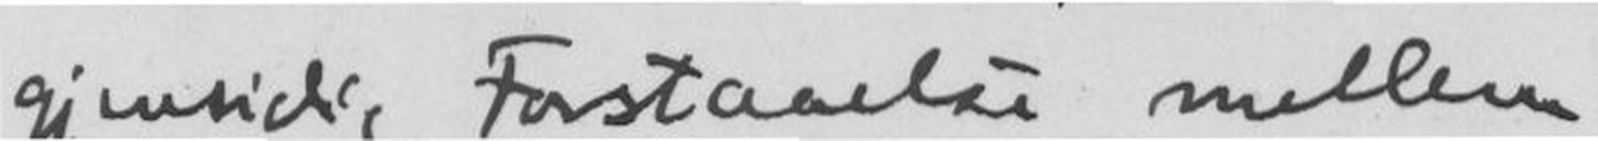gjensidig Forstaaelse mellem
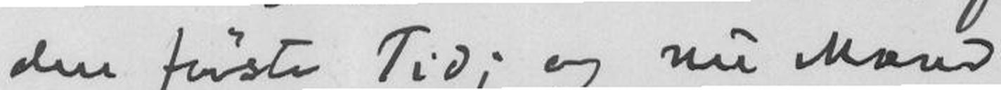den første Tid; og nu Mænd
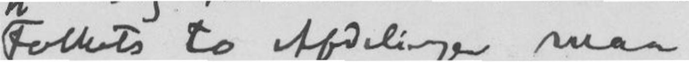Folkets to Afdelinger maa
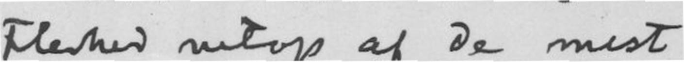Flerhed netop af de mest
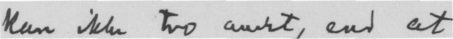kan ikke tro andet, end at
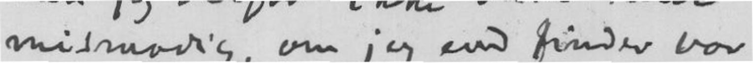mismodig, om jeg end finder vor
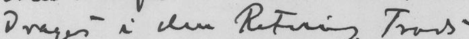drages i den Retning. Trods
